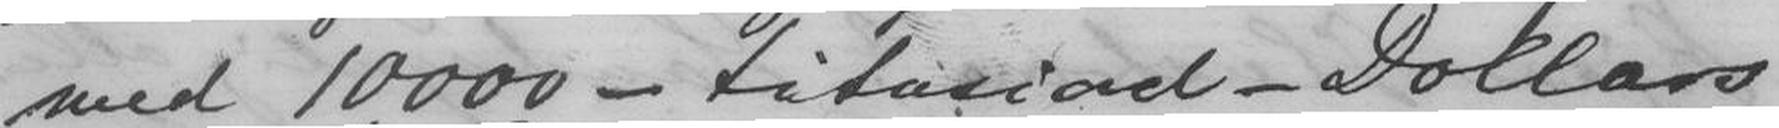med 10,000 - titusind - Dollars
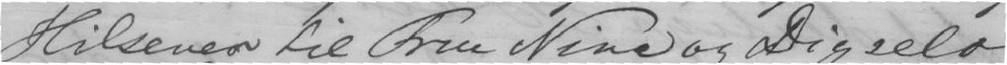Hilsener til Fru Nina og Dig selv
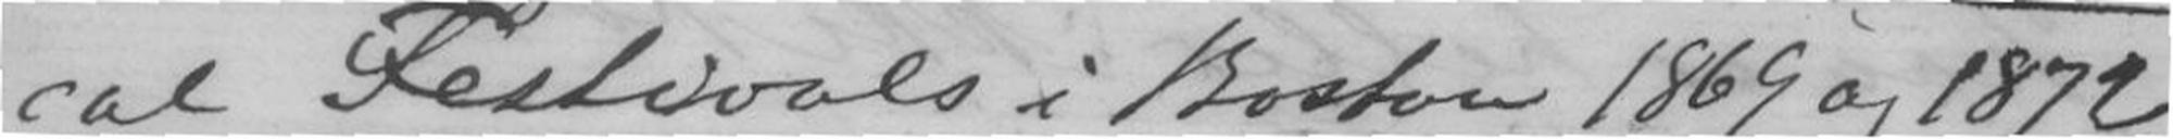cal Festivals i Boston 1869 og 1872
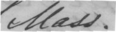Mass.
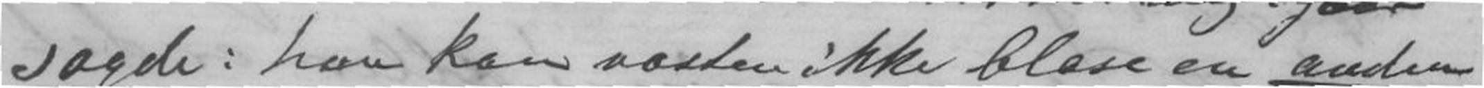sagde: han kan næsten ikke blæse en anden
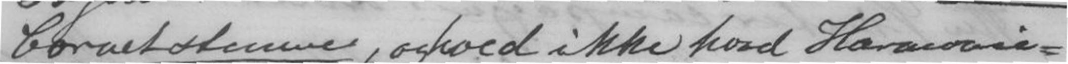Cornetstemme, og hved ikke hvad Harmoni-
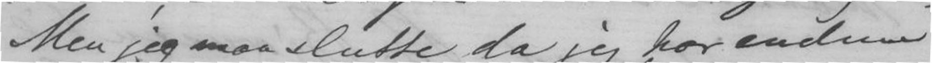Men jeg maa slutte da jeg har endnu
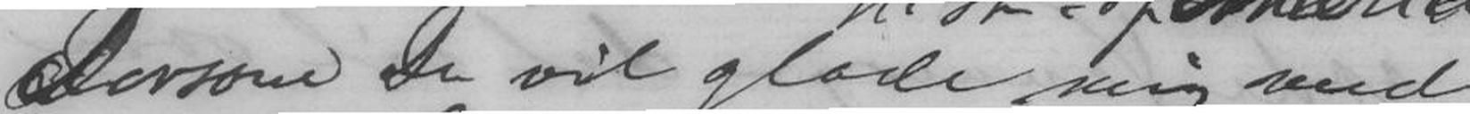Dersom Du vil glade mig med
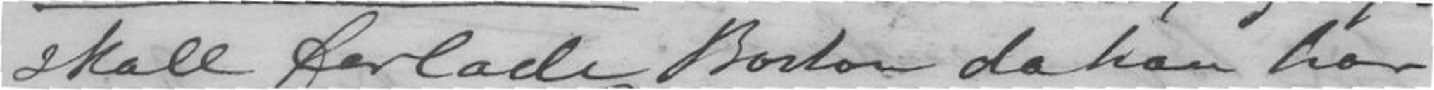skall forlade Boston da han har
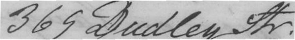369 Dudley Str.
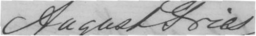August Fries
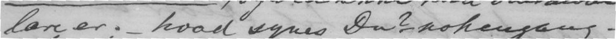lære er, - hvad synes Du? nok engang.
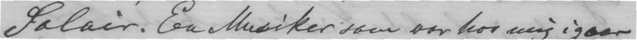Salair. En Musiker som var hos mig igaar
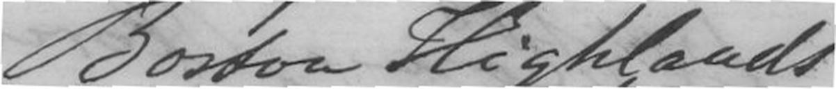Boston Highlands
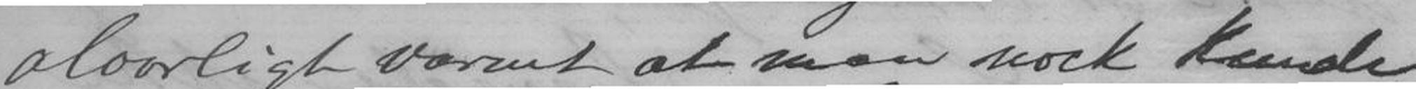alvorligt varmt at man nock kunde
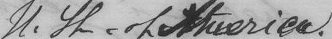U. St. of America.
